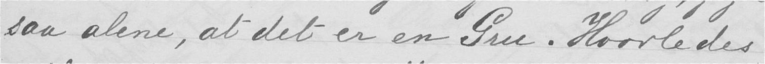saa alene, at det er en Gru. Hvorledes
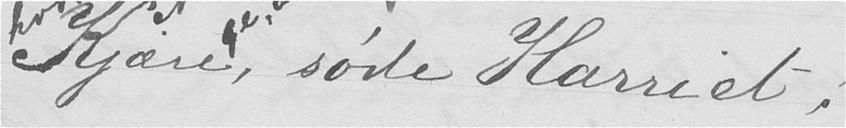Kjære, søde Harriet!
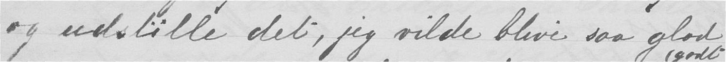og udstille det, jeg vilde blive saa glad
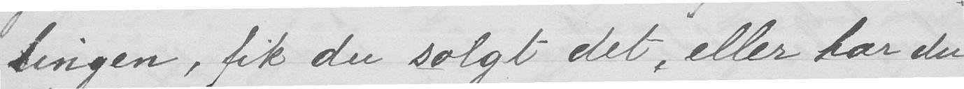lingen, fik du solgt det, eller har du
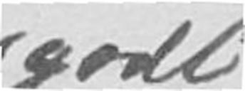godt
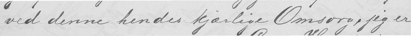ved denne hendes kjærlige Omsorg, jeg er
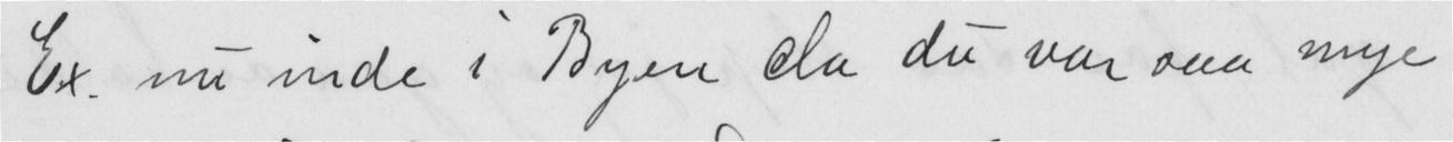Ex. nu inde i Byen da du var saa mye
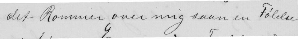det Kommer over mig saan en Følelse
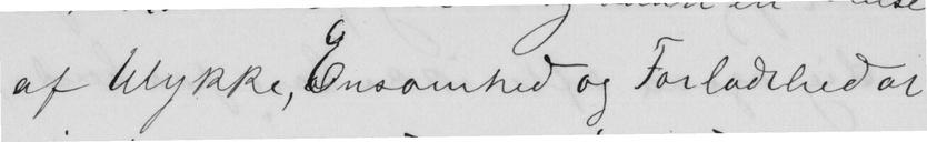af Ulykke, Ensomhed og Forladshedat
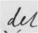det
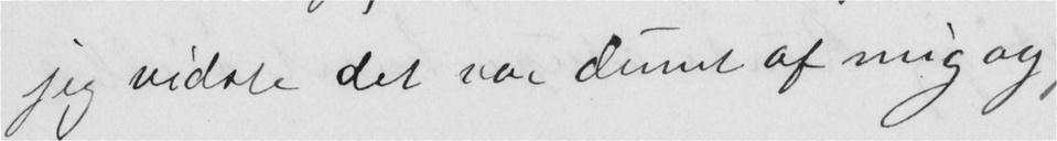jeg vidste det var dumt af mig og jeg,
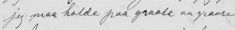jeg maa holde paa graate aa graate
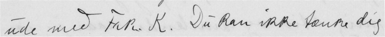ude med Frk K. Du kan ikke tænke dig
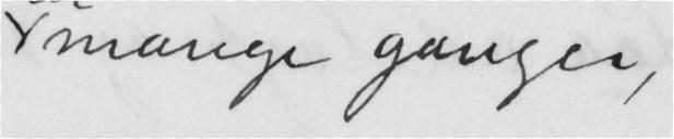mange ganger,
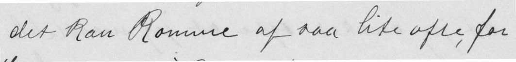det kan Komme af saa lite ofte, for
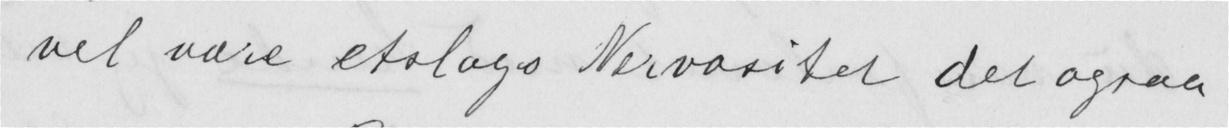vel være etslags Nervøsitet det ogsaa
